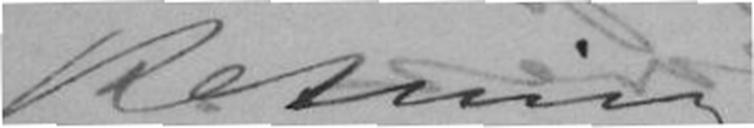hengivne
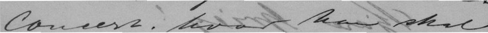Concert; hvori han skal
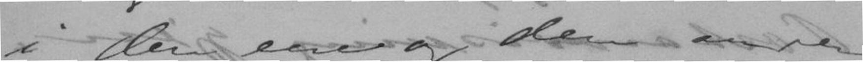forbliver jeg Deres
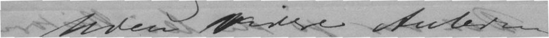Harald Ibsen
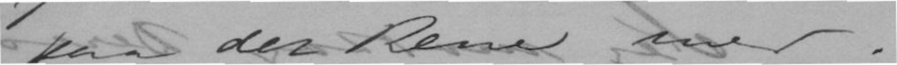paa det Rene med.
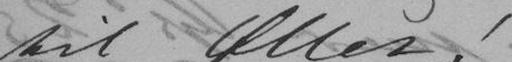Øllet!
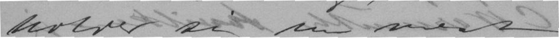holder sig nu mest til
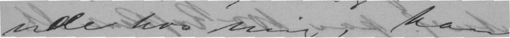ude hos mig, han
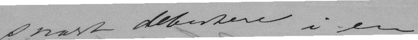snart debutere i en
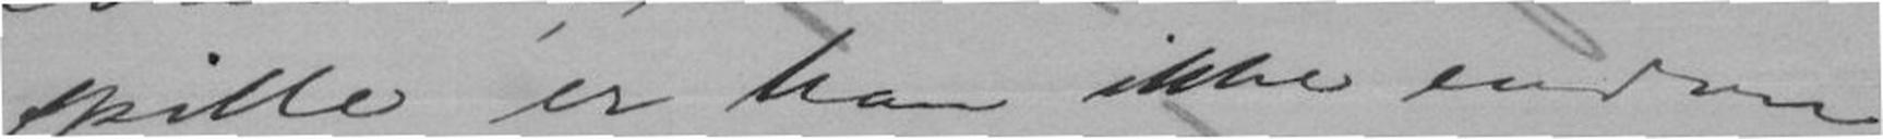spille er han ikke endnu
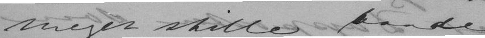for idag
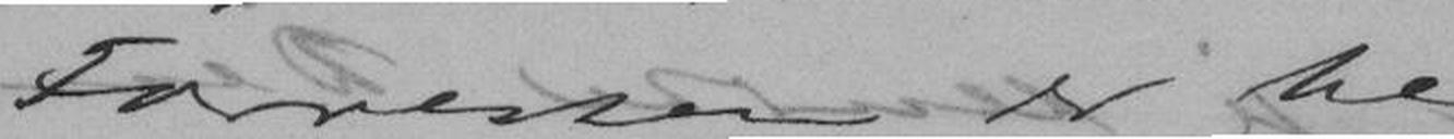Uden videre Anledning
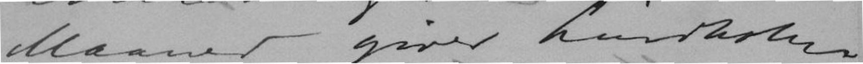Maaned giver Lindholm
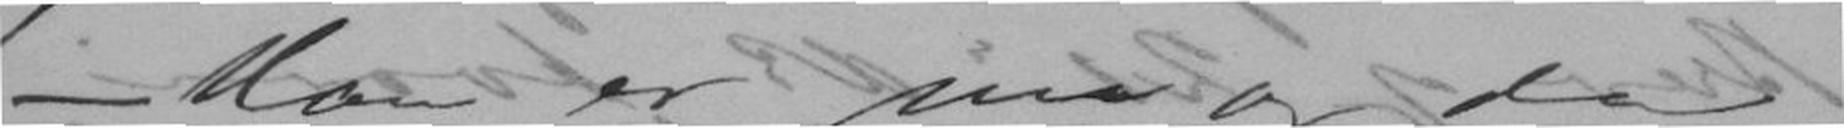Han er nu og da
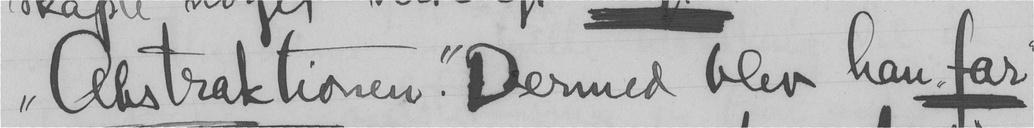"Abstraktionen". Dermed blev han "far"
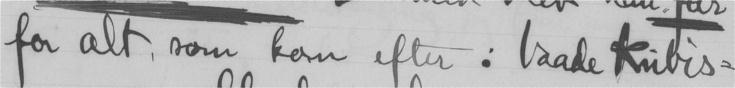for alt, som efter: baade kubis-
